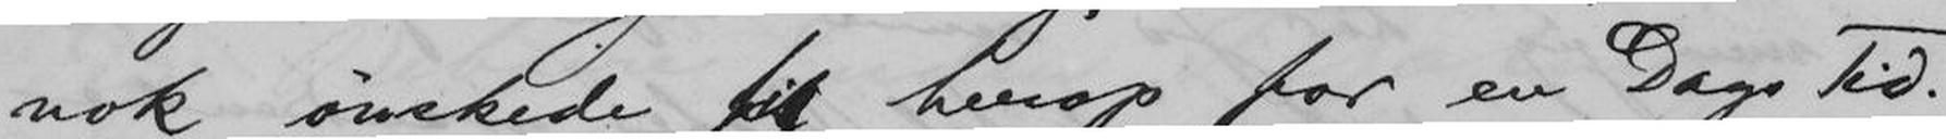nok ønskede sig herop for en Dags Tid.
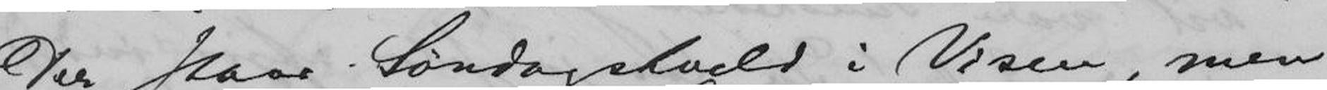Der staar Søndagskveld i Visen, men
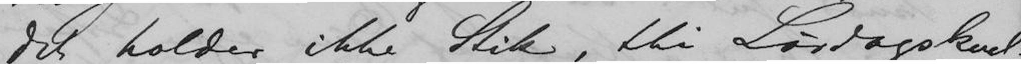det holder ikke Stik, thi Lørdagskvel-
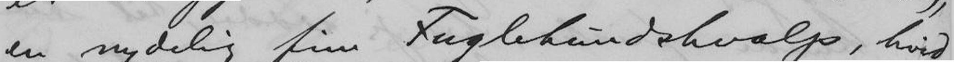en nydelig fin Fuglehundshvalp, hvid
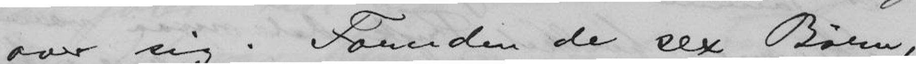over sig. Foruden de sex Børn,
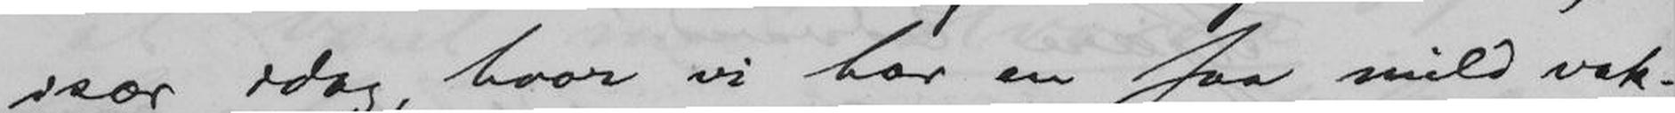især idag, hvor vi har en saa mild vak-
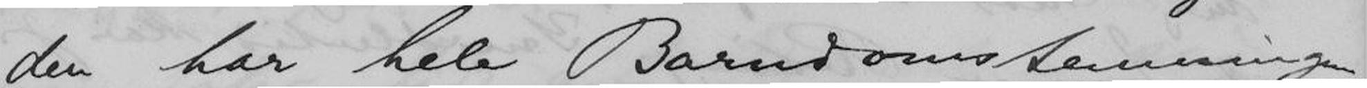den har hele Barndomstemningen
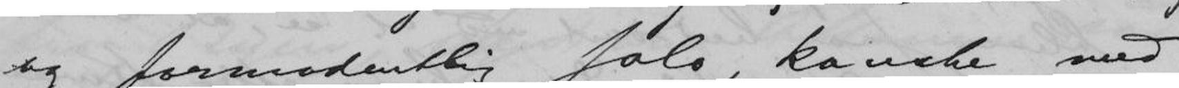og formodentlig solo, kanske med
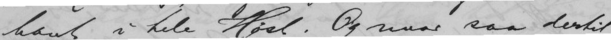havt i hele Høst. Og naar saa dertil
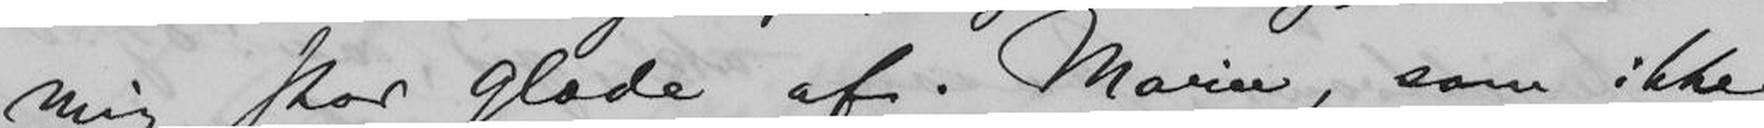mig stor glæde af. Marie, som ikke
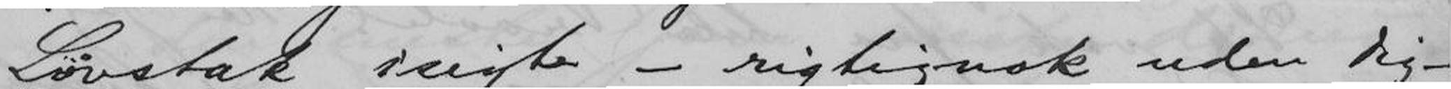Løvstak isigte - rigtignok uden Dig -
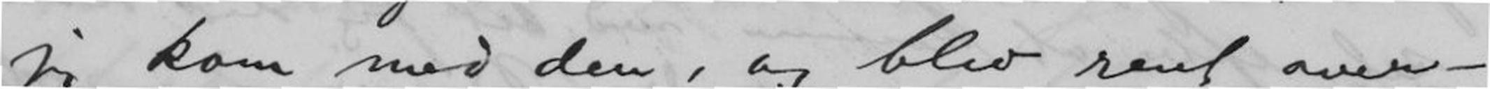jeg kom med den, og blev rent over-
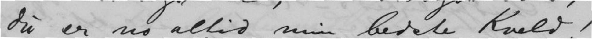du er no altid min bedste Kveld!
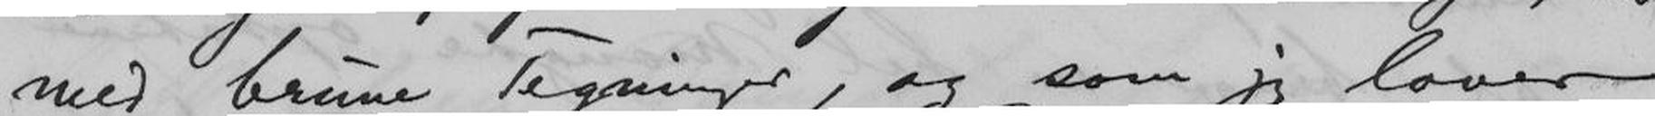med brune Tegninger, og som jeg lover
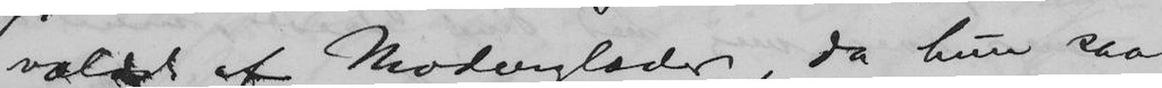vældet af Moderglæder, da hun saa
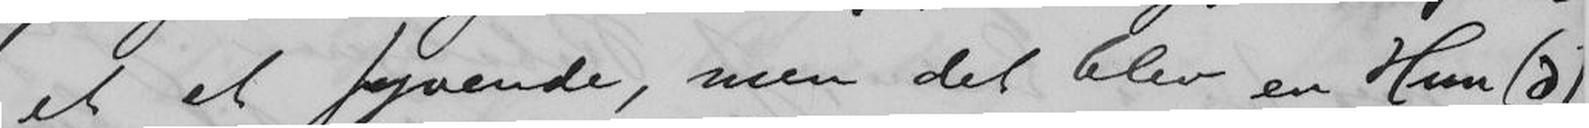et et syvende, men det blev en Hun(d),
